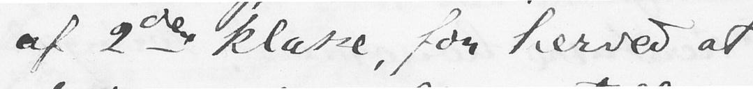af 2den klasse, for herved at
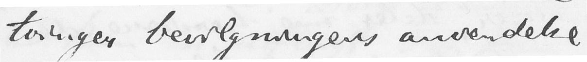tvinger bevilgningens anvendelse
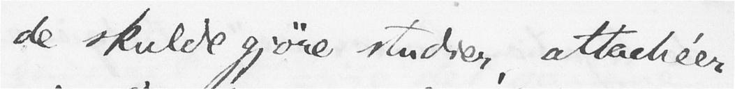de skulde gjøre studier, attachéer
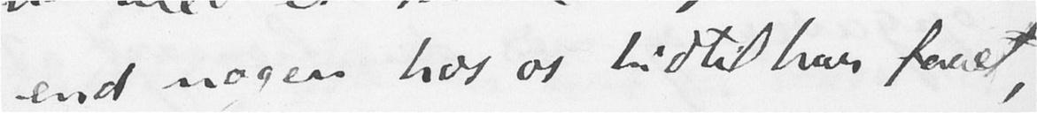end nogen hos os hidtil har faaet,
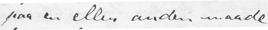paa en eller anden maade
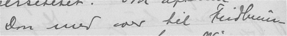Don med over til Fridheim -
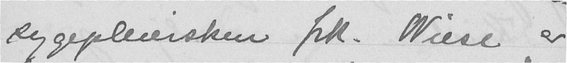sygepleiersken frk. Wiese er
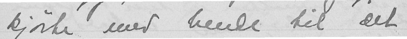kjørte med hende til det
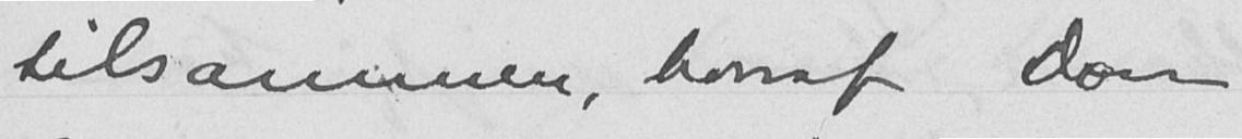tilsammen, hvoraf Don
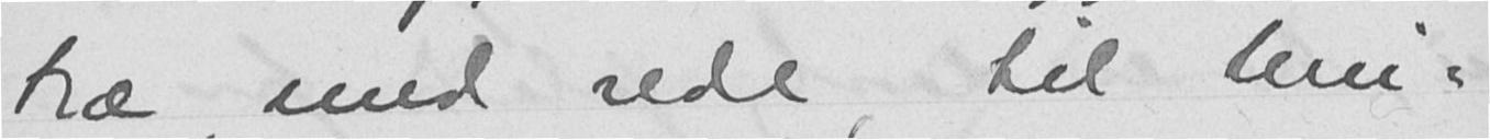træ, med rede, til Uni-
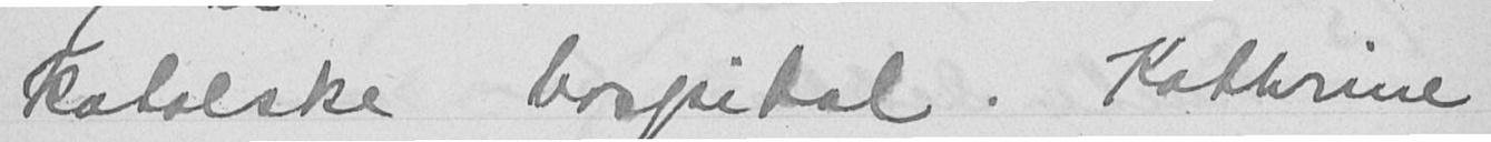katolske hospital. Kathrine
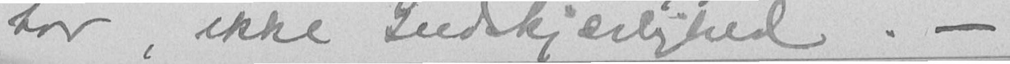har, ikke Gudskjærlighed. -
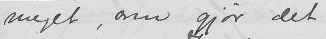meget, som gjør det
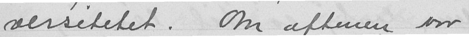versitetet. Om aftenen var
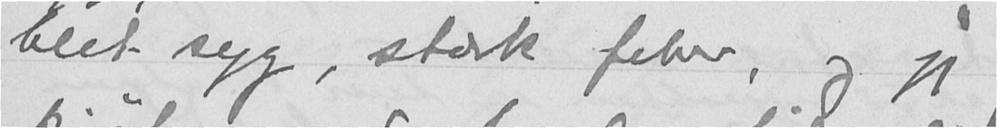blit syg, stærk feber, og jeg
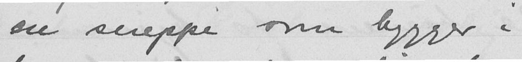en sneppe som bygger i
